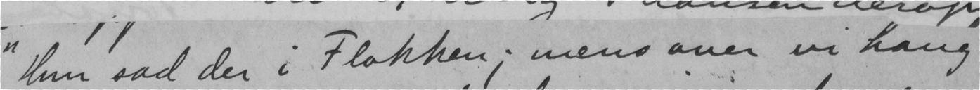"Hun sad der i Flokken; mens over vi hang
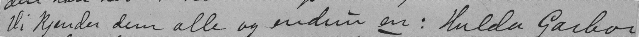Vi kjender dem alle og endnu en: Hulda Garborg
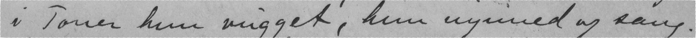i Toner hun vugget, hun nynned og sang.
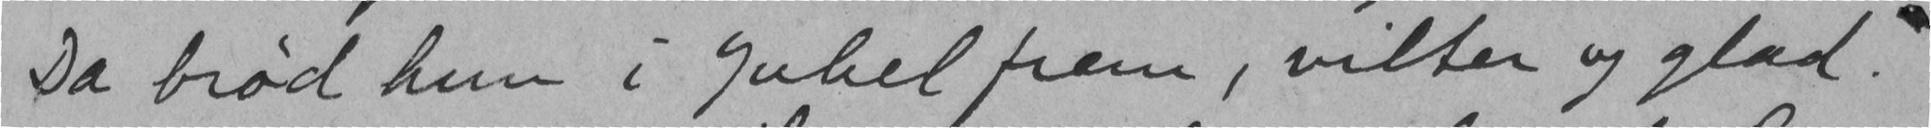Da brød hun i Jubel frem, vilter og glad."
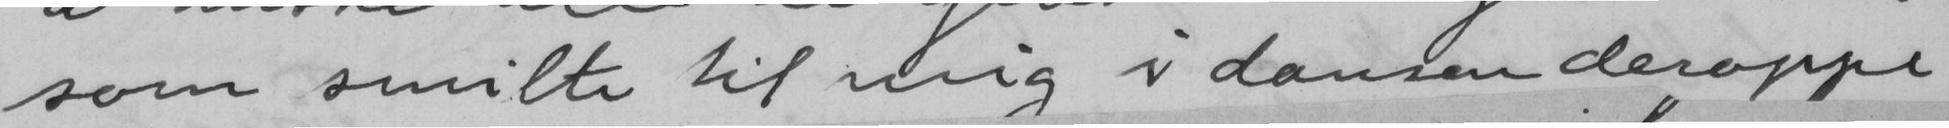som smilte til mig i dansen deroppe
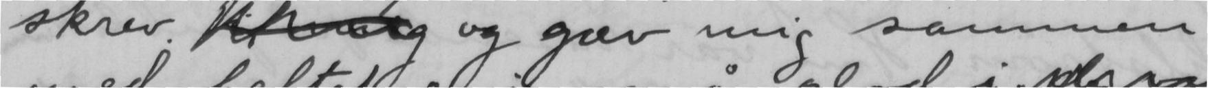skrev.  og gav mig sammen
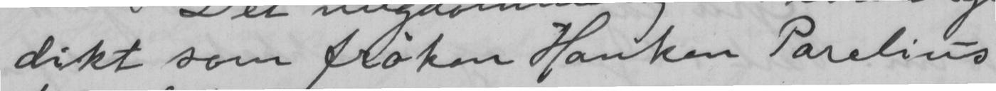dikt som frøken Hanken Parelius
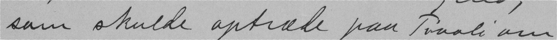som skulde optræde paa Tivoli om
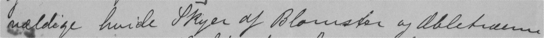vældige hvide Skyer af Blomster og Æbletræerne
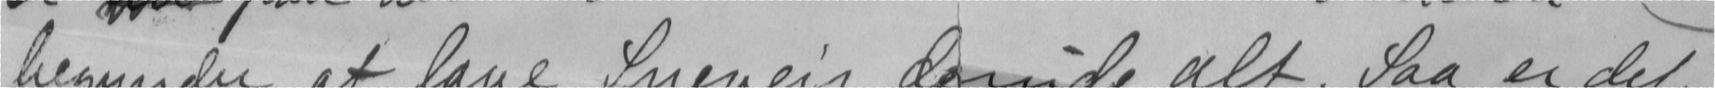begunder at lave Sneveir derinde alt. Saa er det
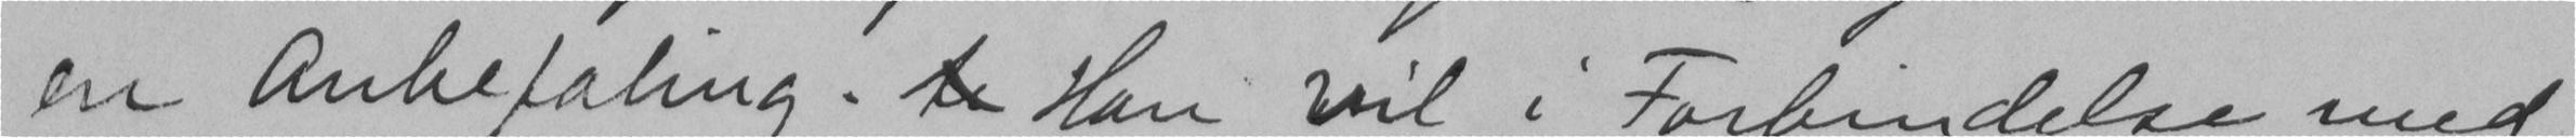en Anbefaling.  Han vil i Forbindelse med
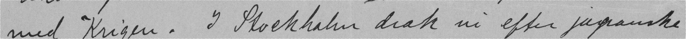med Krigen. I Stockholm drak vi efter japanske
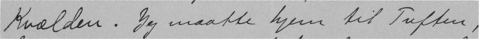Kvælden. Jeg maatte hjem til Tuften,
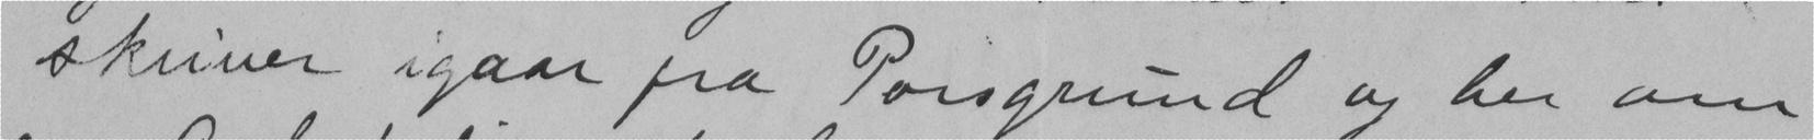skriver igaar fra Porsgrund og her om
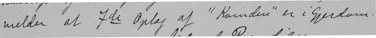melder at 7de Oplag af "Kvinden" er i Gjendom.
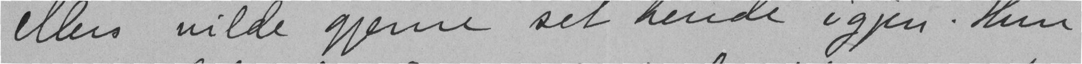ellers vilde gjerne set hende igjen. Hun
